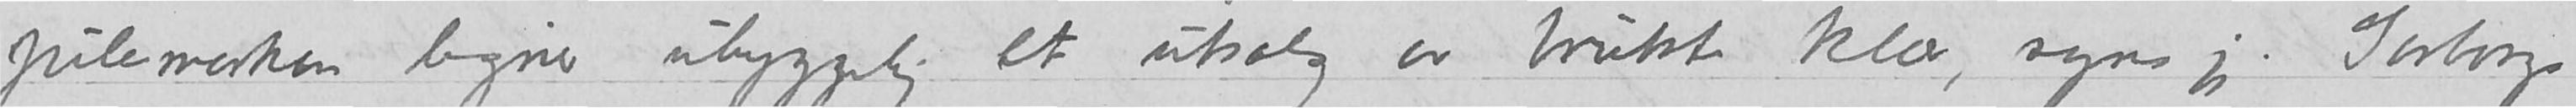julemarken ligner uhyggelig et utsalg av brukte klær, syns jeg. Garborgs
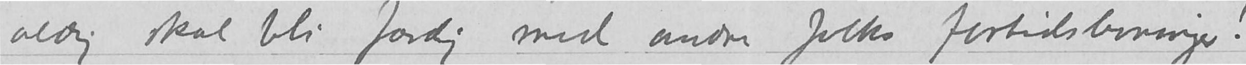aldrig skal bli færdig med andre folks fortidslevninger!
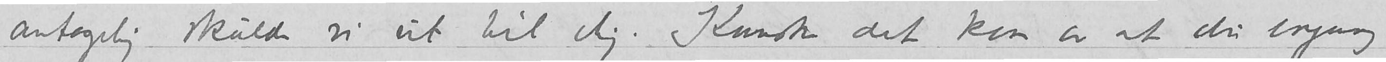antagelig skulde vi ut til dig. Kanske det kom av at du engang
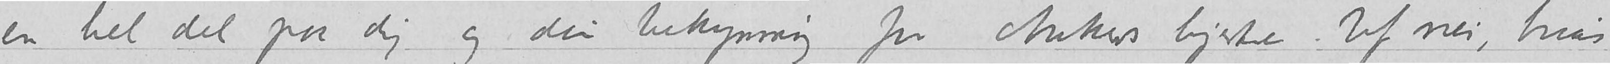en hel del paa dig og din bekymring for Ankers hjerte. Uf nei, hvis
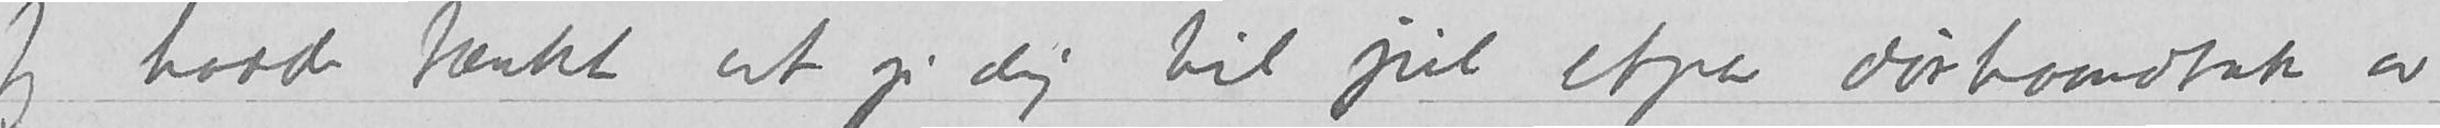Jeg hadde tænkt at gi dig til jul etpar dørhaandtak av
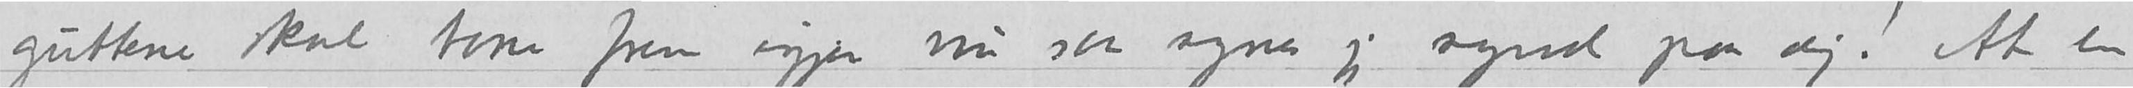guttene skal tone frem igjen nu saa synes jeg synd paa dig! At en
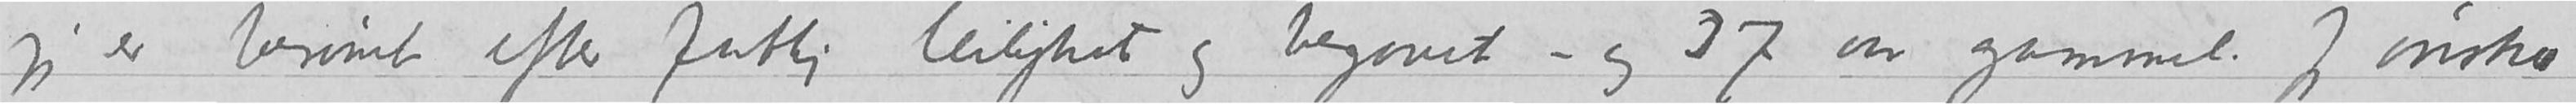jeg er berømt efter fattig leilighet og begavet – og 37 aar gammel. Jeg ønsker
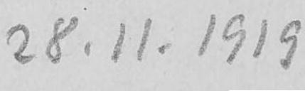28.11.1919
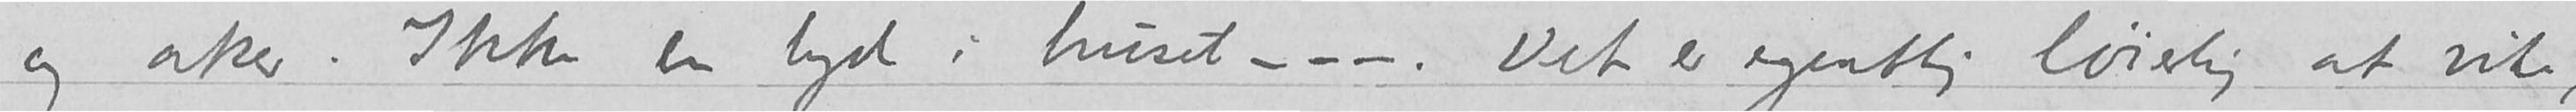og aker. Ikke en lyd i huset – – –. Det er egentlig løierlig at vite,
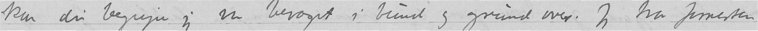kan du begripe jeg var bevæget i bund og grund over. Jeg tror forrestem
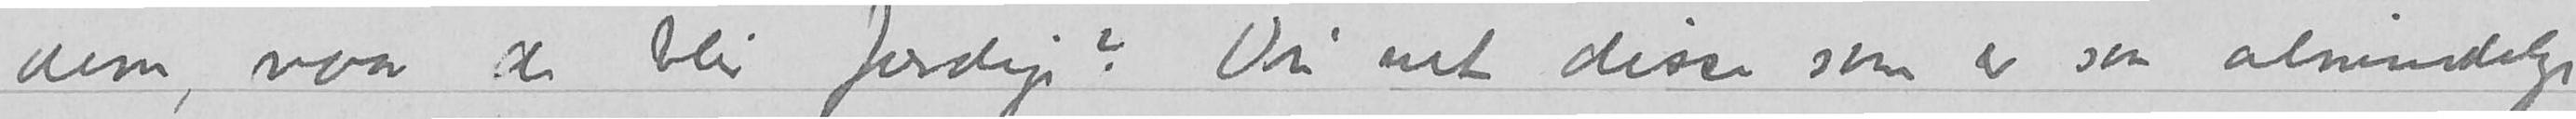dem, naar de blir ferdige? Du vet disse som er saa almindelige
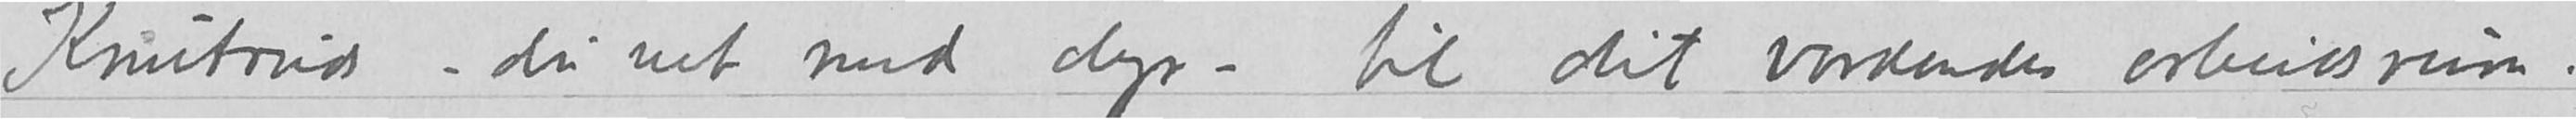Knutruds – du vet med dyr – til dit vordende arbeidsrum.
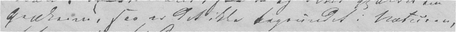Grækerne, saa er det ikke begrundet i Naturen,
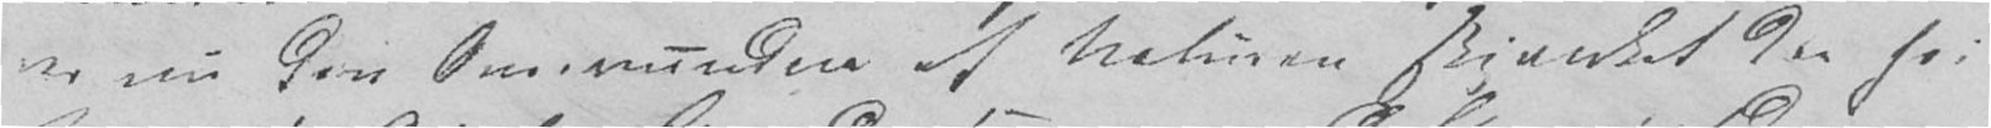er nu den Overvundne af Naturen skjænket den fri
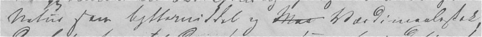Natur som Byttemiddel og Maa Værdimaalestok
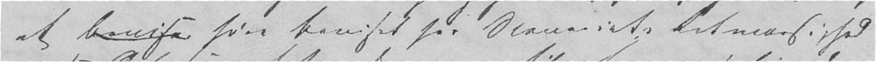at bevise føre Beviset for Slaveriets Retmæssighed
